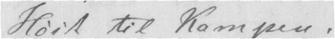Høit til Kampen.
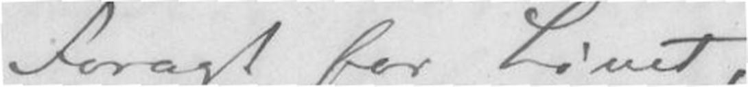Foragt for Livet,
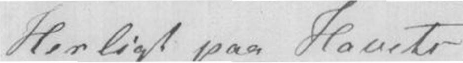Herligt paa Havets
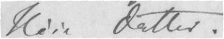Høie Datter.
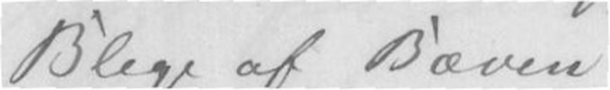Blege af Bæven
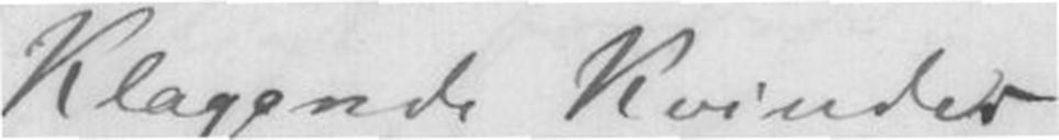Klagende Kvinder
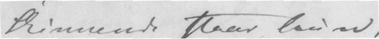Skinnende staar laun,
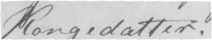Kongedatter.
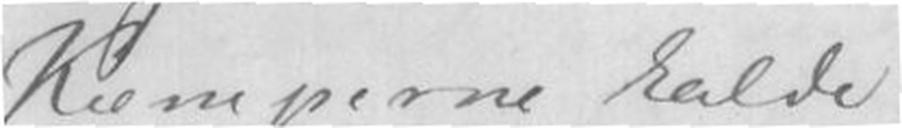Kæmperne kalde
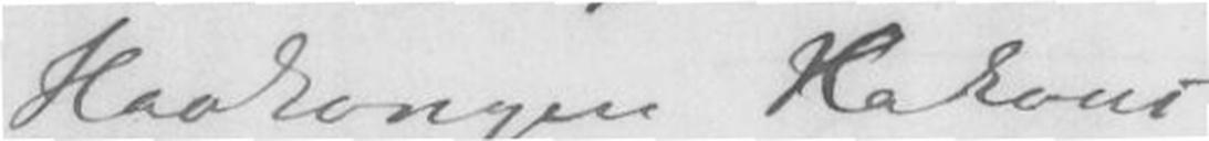Havkongen Hakons
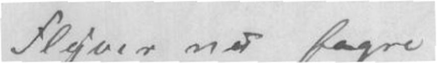Flyver med fagre
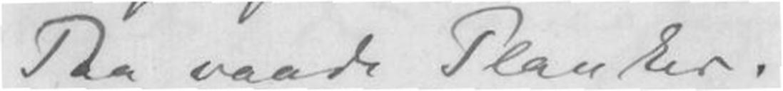Paa vaade Planker.
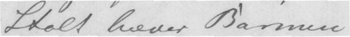Stolt hæver Barmen
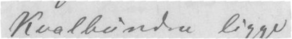Kvalbundne ligge
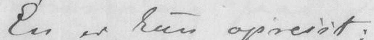En er kun opreist;
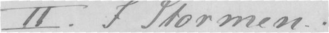II. I Stormen.
